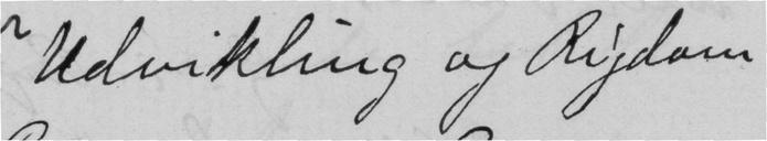Udvikling og Rigdom
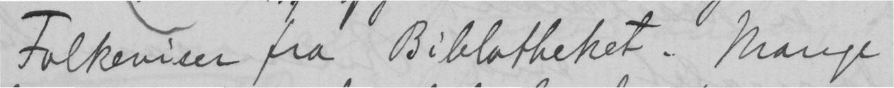Folkeviser fra Biblotheket. Mange
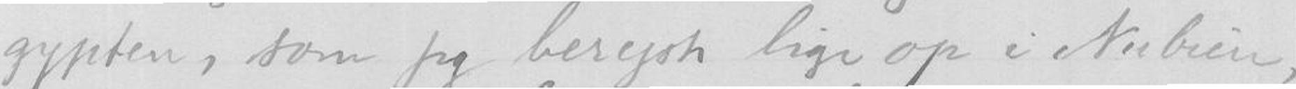gypten, som jeg berejste lige op i Nubien,
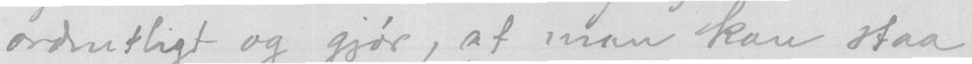ordentligt og gjør, at man kan staa
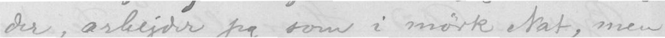der, arbejder jeg som i mørke Nat, men
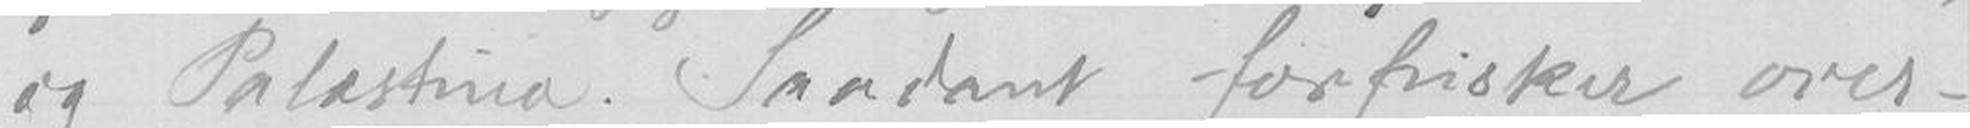og Palestina. Saadant forfrisker over-
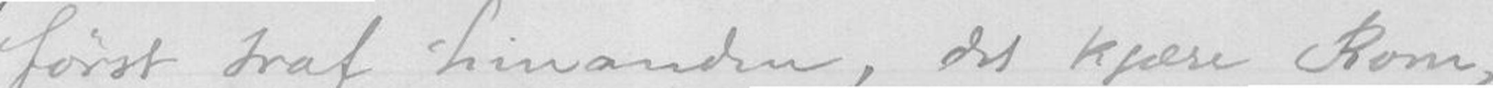først traf hinanden, det kjære Rom,
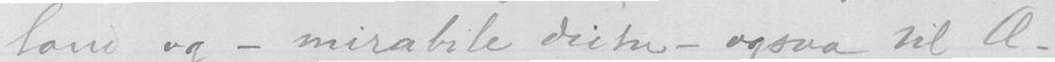land og - mirabile diche - ogsaa til Æ-
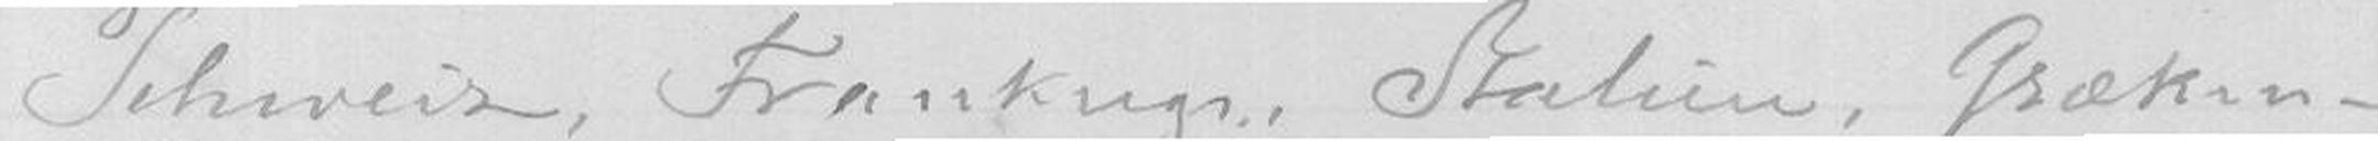Schweiz, Frankrige, Italien, Græken-
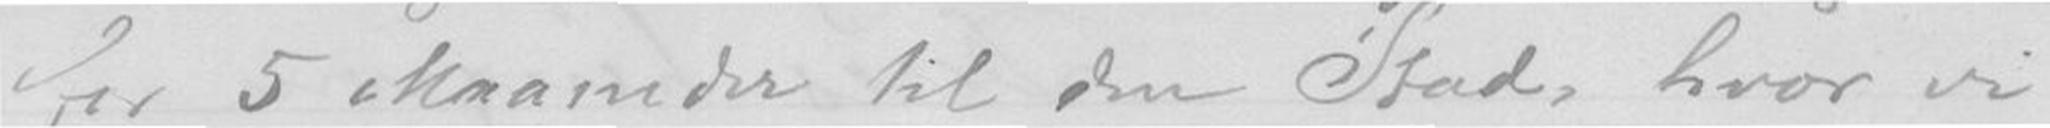for 5 Maaneder til den Stad, hvor vi
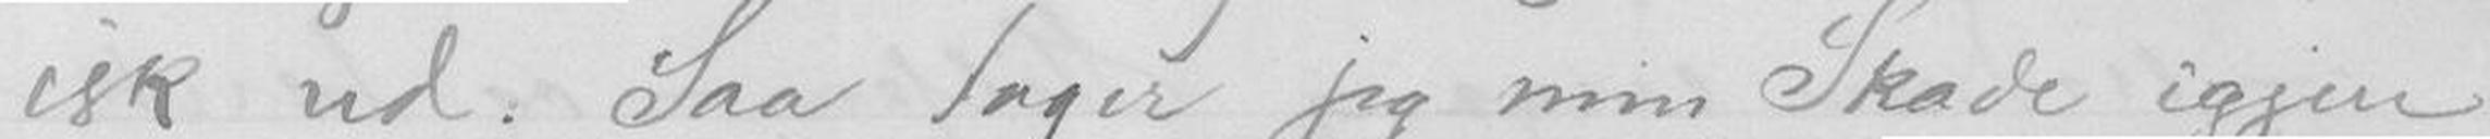isk ud. Saa tager jeg min Skade igjen
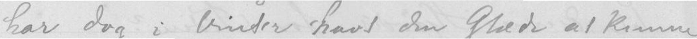har dog i Vinter havt den Glæde at kunne
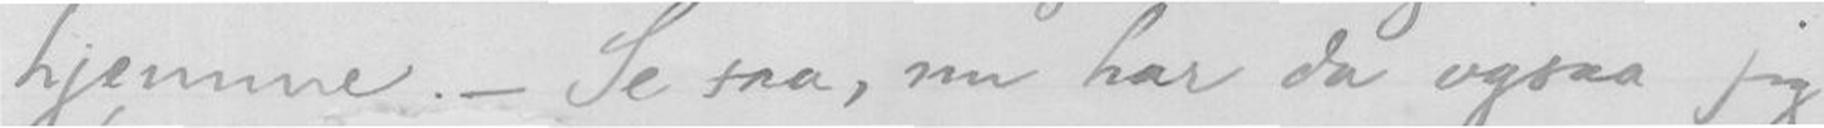hjemme. - Se saa, nu har da ogsaa jeg
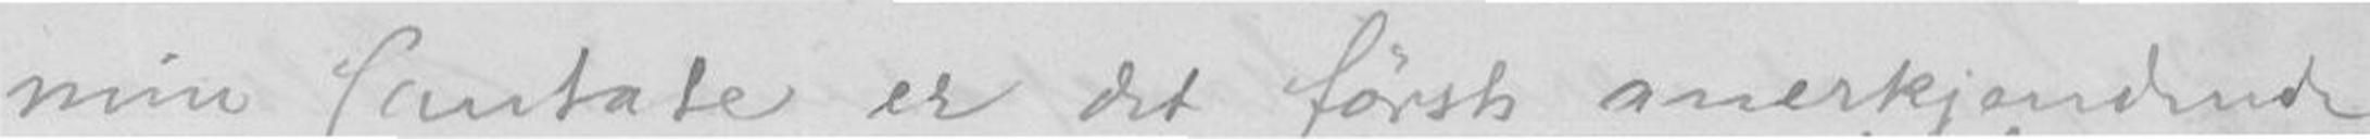min Cantate er det første anerkjendende
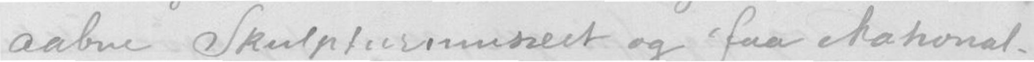aabne Skulpturmusæet og faa National-
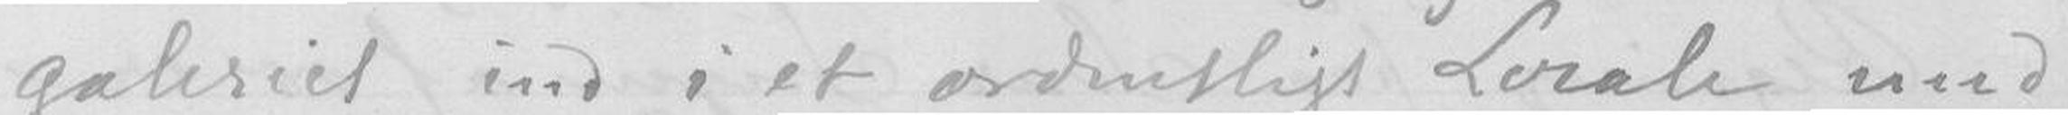galeriet ind i et ordentligt Locale med
